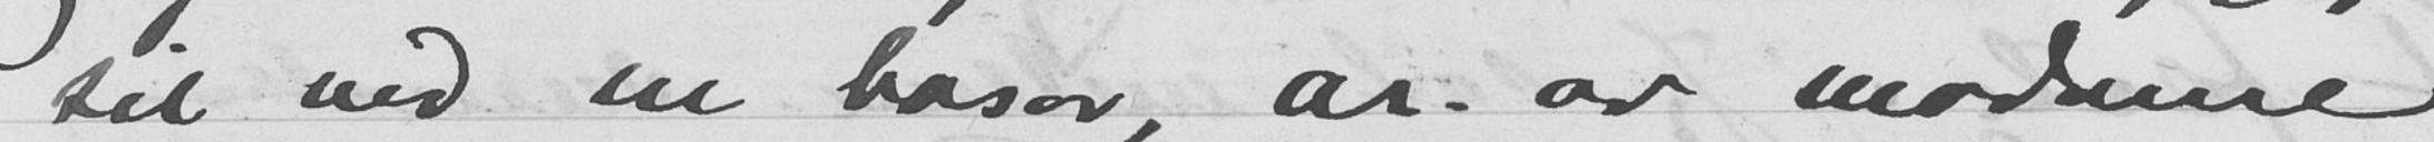til ved en basar, ar. av moderne
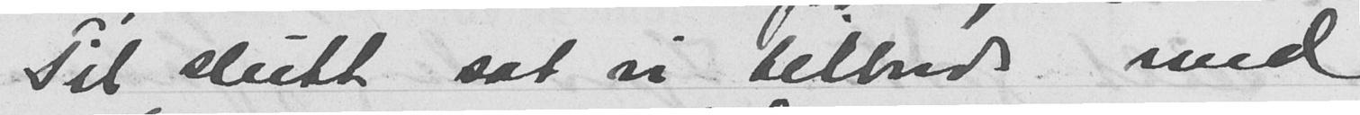Til slutt sat vi tilbords med
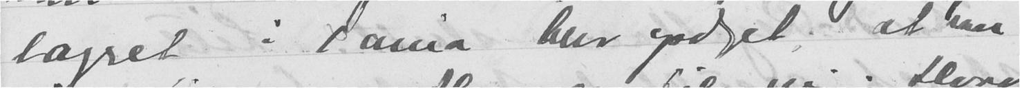lagret i Xania blev opdaget, at han
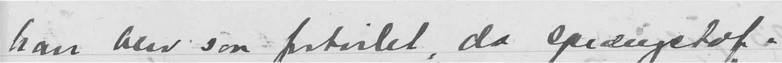han blev saa fortvilet, da sprængstof-
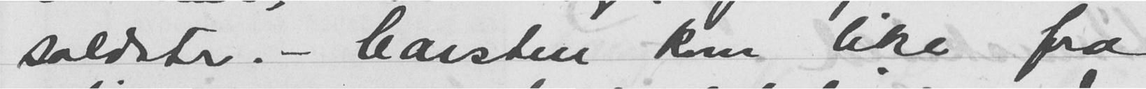soldater. - Carsten kom like fra
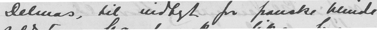Delmas, til indtægt for franske blinde
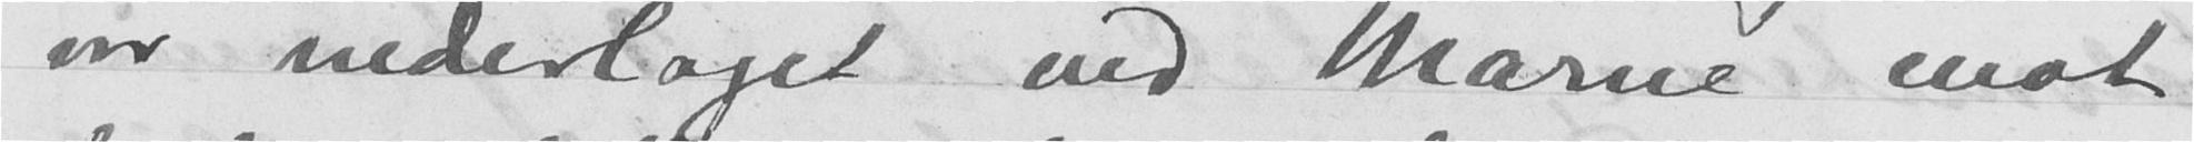var nederlaget ved Marne mot
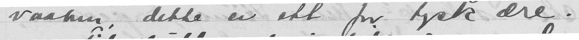vaaben, dette er ett for tysk ære.
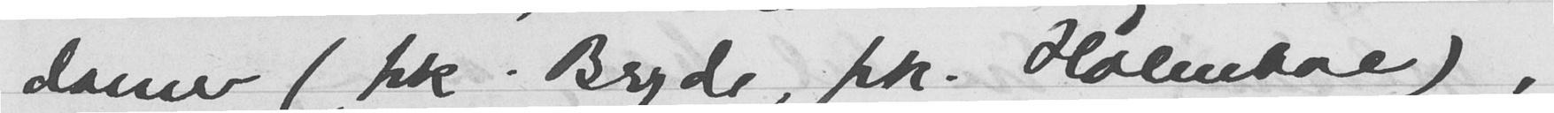damer (frk. Bryde, frk. Holmboe),
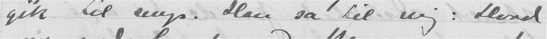gik til sengs. Han sa til mig: Hvad
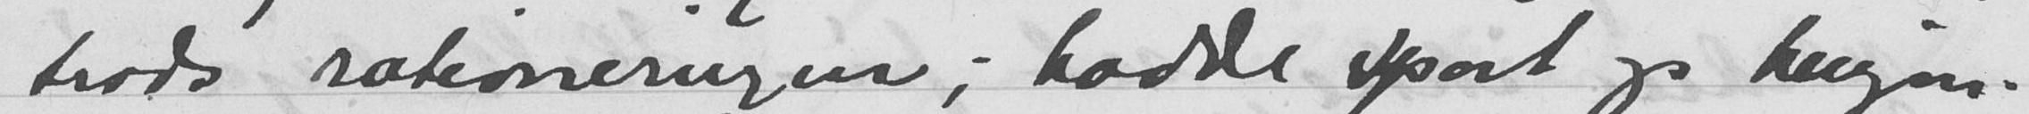trods rationeringen; hadde sport og kujon.
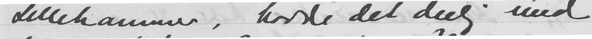Lillehammer, hadde det deilig med
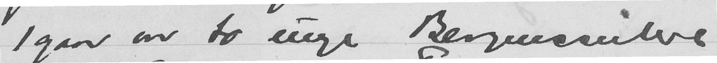Igaar var to unge Bergensseilere
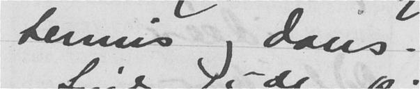tennis og dans.
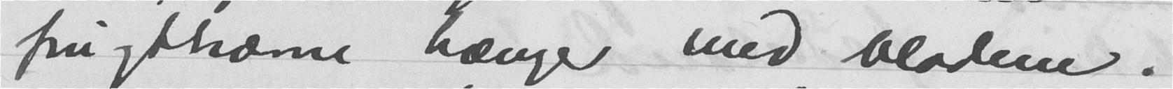frugttrærne hænger med bladene.
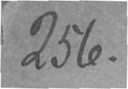256.
